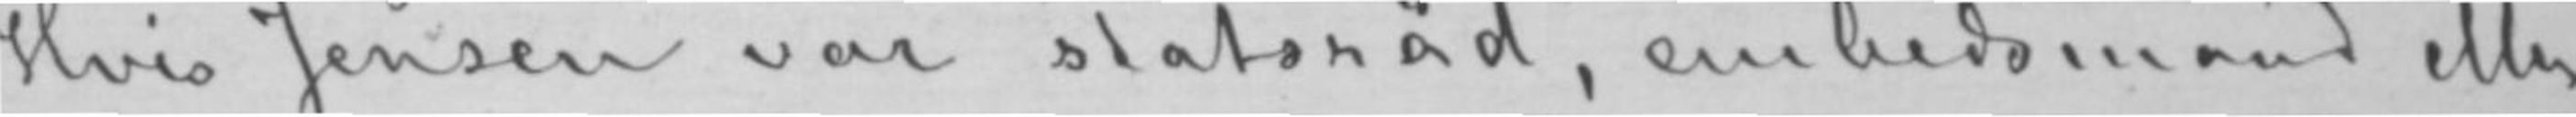Hvis Jensen var statsråd, embedsmand eller
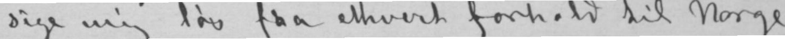sige mig løs fra ethvert forhold til Norge
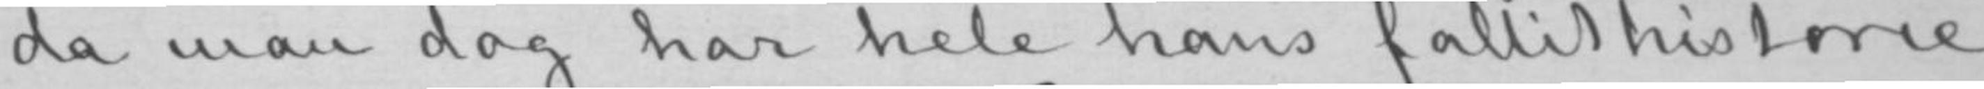da man dog har hele hans fallithistorie
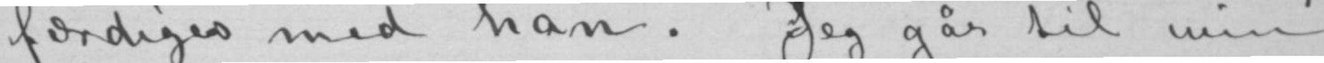færdiges med hån. Jeg går til min
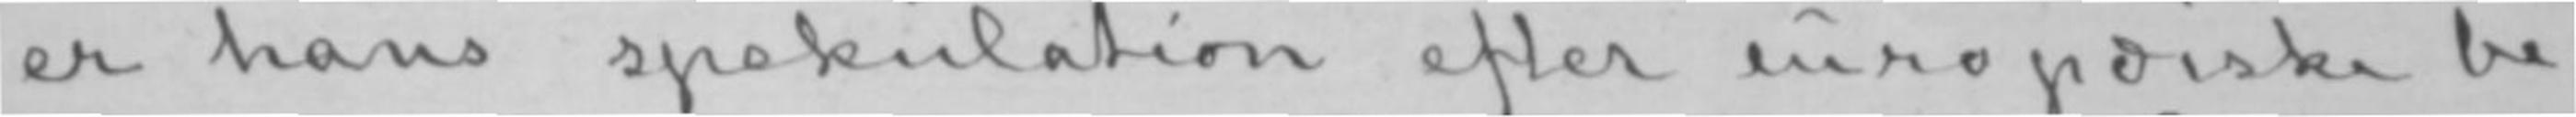er hans spekulation efter europæiske be-
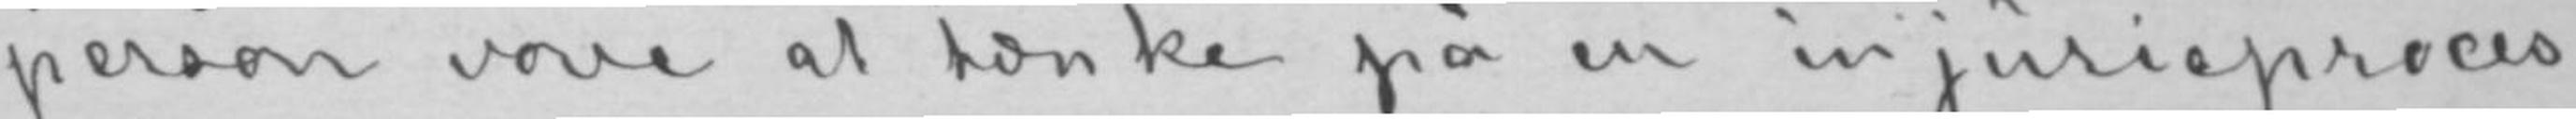person vove at tænke på en injurieproces,
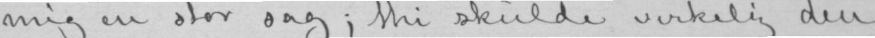mig en stor sag; thi skulde virkelig den
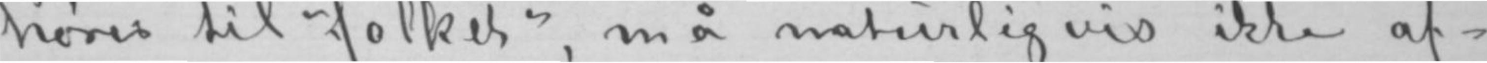hører til «folket», må naturligvis ikke af-
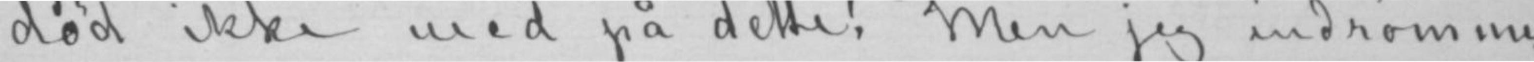død ikke med på dette! Men jeg indrømmer
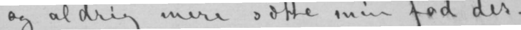og aldrig mere sætte min fod der.
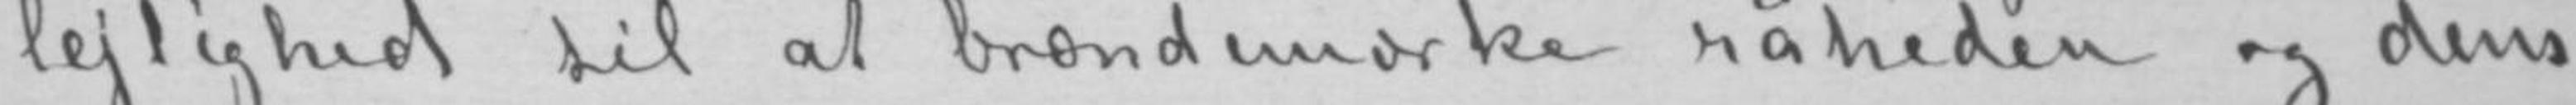lejlighed til at brændemærke råheden og dens
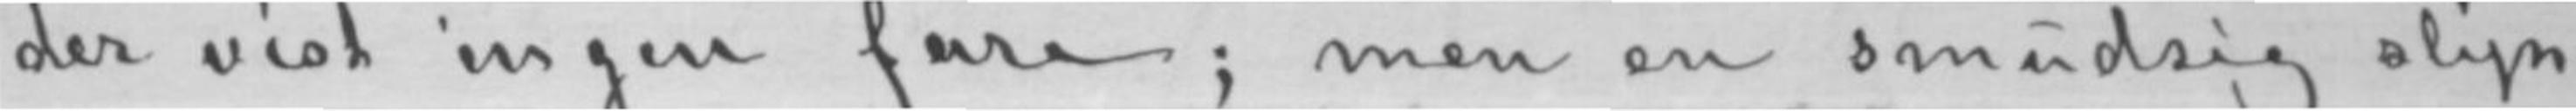der vist ingen fare; men en smudsig slyn-
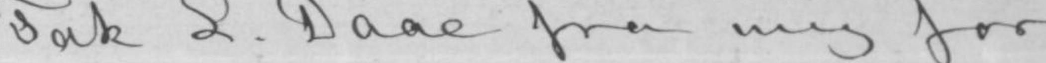Tak L. Daae fra mig for
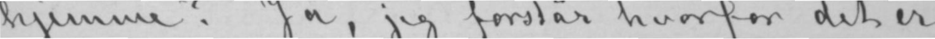hjemme? Ja, jeg forstår hvorfor det er
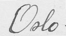Oslo.
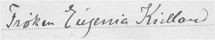Frøken Eugenia Kielland
